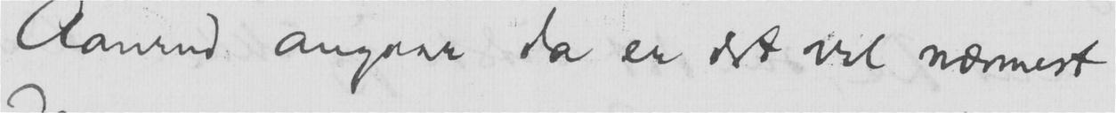Aanrud angaar da er det vel nærmest
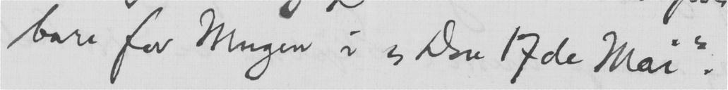bare for Mugen i "Den 17de Mai".
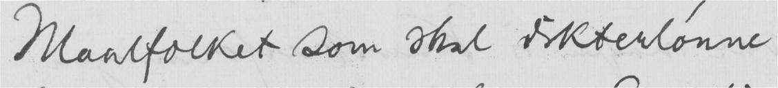Maalfolket som skal dikterlønne
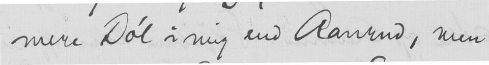mere Døl i mig end Aanrud, men
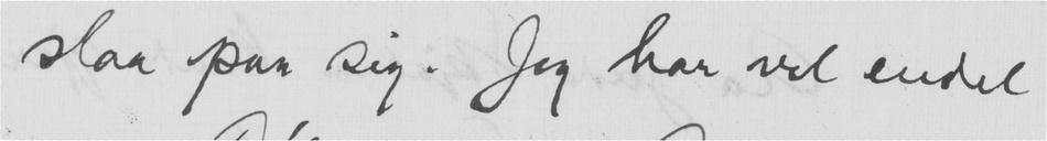slaa paa sig. Jeg har vel endel
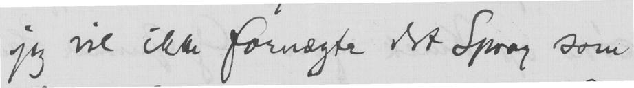jeg vil ikke fornægte det Sprog som
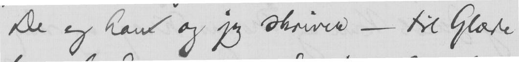De og han og jeg skriver - til Glæde
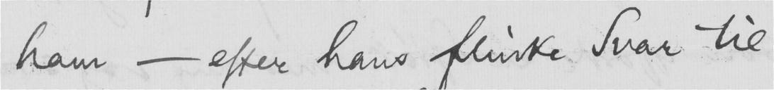ham - efter hans flinke Svar til
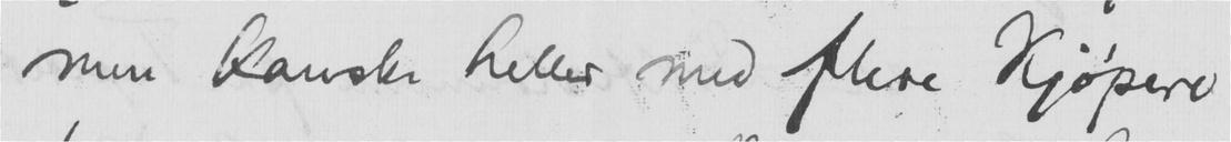men kanske heller med flere Kjøpere
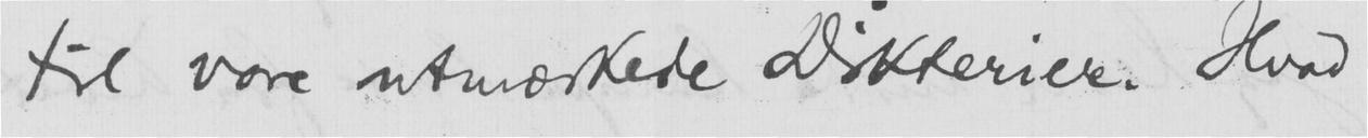til vore utmærkede Dikterier. Hvad
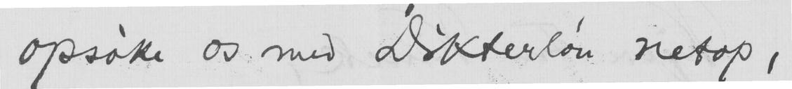opsøke os med Dikterløn netop,
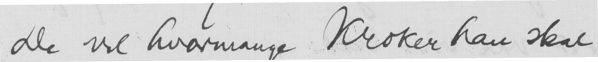De vel hvormange Kroker han skal
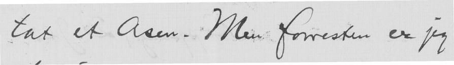tat et Asen. Men forresten er jeg
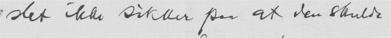slet ikke sikker paa at den skulde
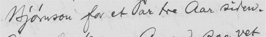Bjørnson for et Par tre Aar siden.
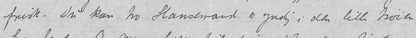frisk. Du kan tro Hansemand er yndig i den lille trøien
